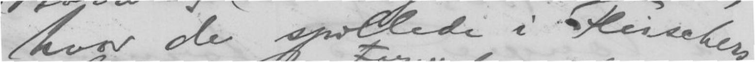hvor de spillede i Fleischers
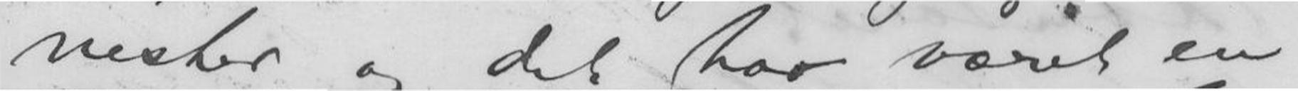nesker og det har været en
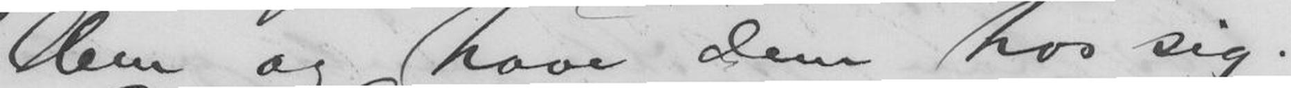Dem og have dem hos sig.
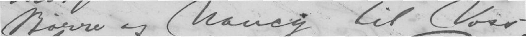Børre og Nancy til Voss,
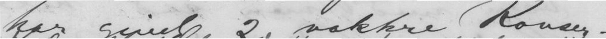har givet 2 vakkre Konser-
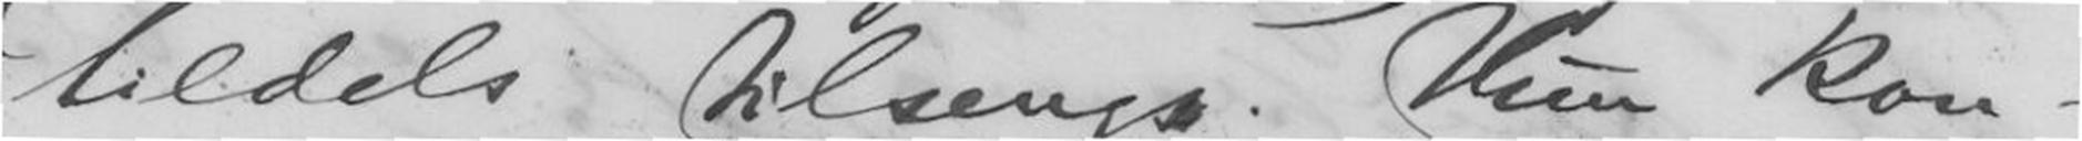tildels tilsengs. Hun kon-
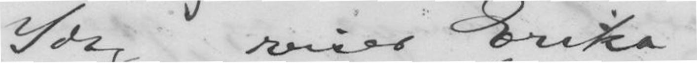Idag reiser Erika og
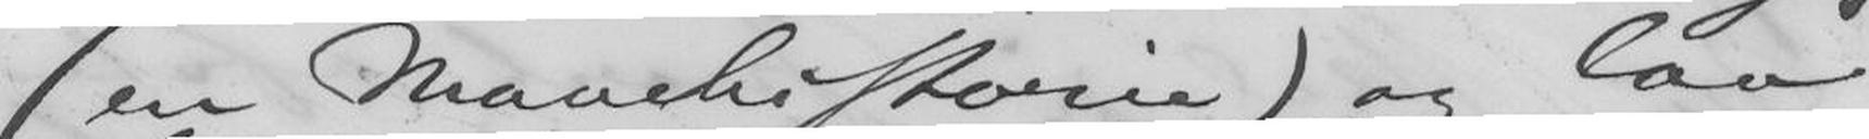(en Mavehistorie) og laa
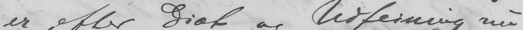er efter Diæt og Udfeining nu
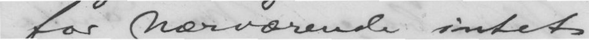jeg for nærværende intet
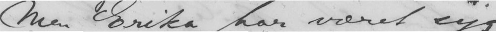Men Erika har været syg
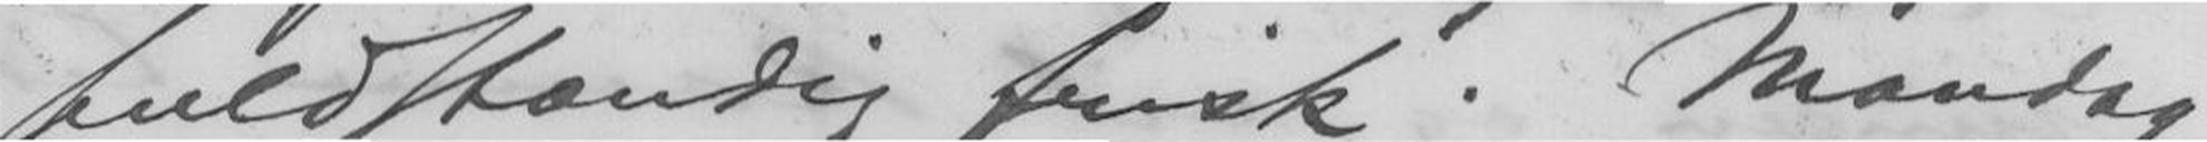fuldstændig frisk. Mandag
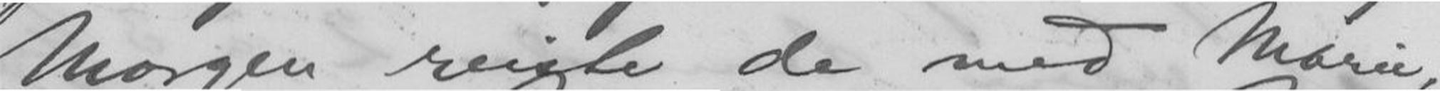Morgen reiste de med Marie,
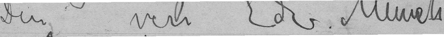Din ven Edv. Munch
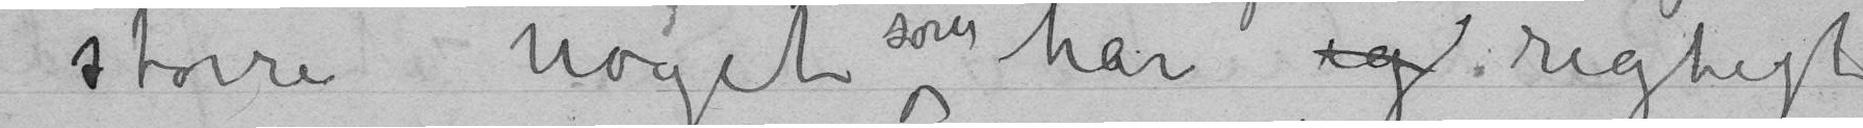større noget som har ig rigtigt
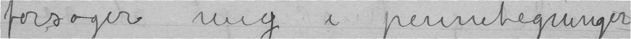forsøger mig i pennetegninger.
